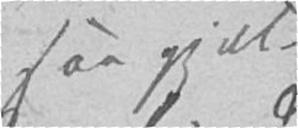saa gjæl-
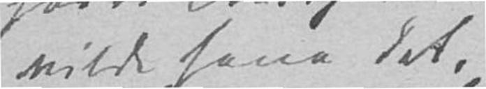vilde have det,
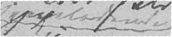tilfredsstillende
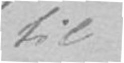til
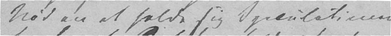Nød an at holde sig Speculationen
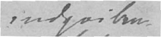indgriben-
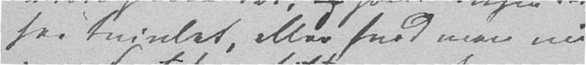har tvivlet, eller hvad man nu
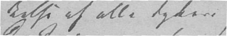kelse af alle dybere
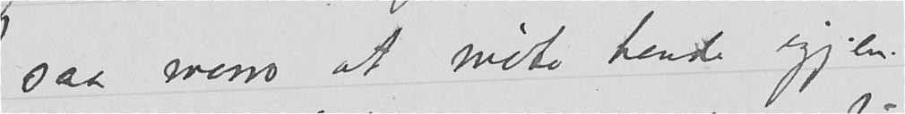saa morro at møte hende igjen.
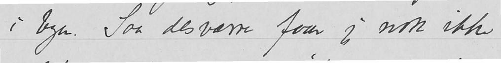i byen. Saa desværre faar jeg nok ikke
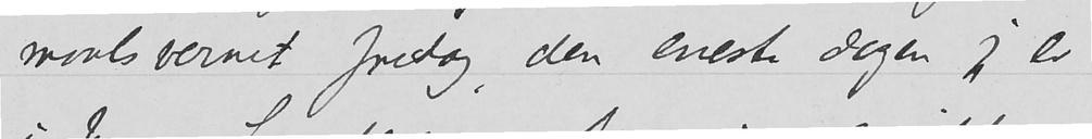maalsvernet fredag, den eneste dagen jeg er
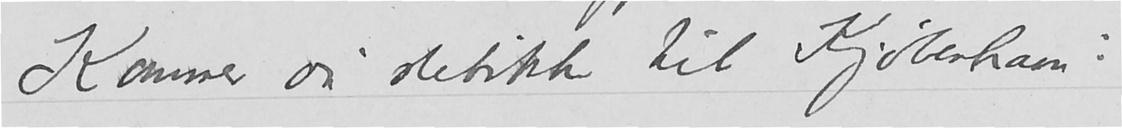Kommer du sletikke til Kjøbenhavn
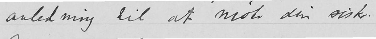anledning til at møte din søster.
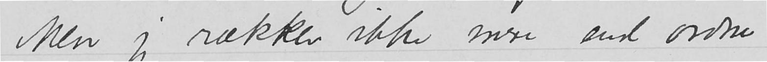Men jeg rækker ikke mere end ordne
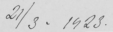21/3 - 1923
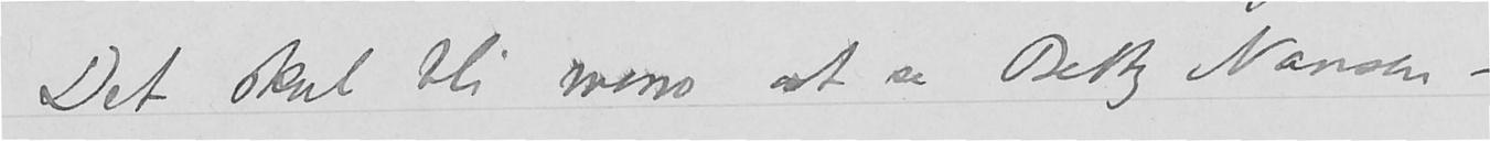Det skal bli morro at se Betty Nansen –
